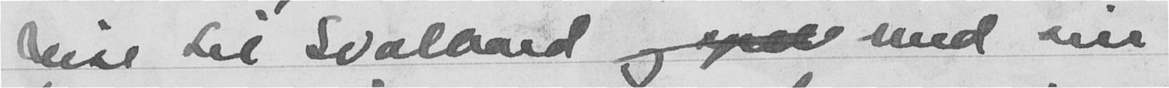reise til Svalbard  med sin
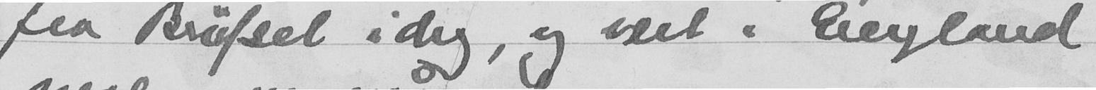fra Brüfsel idag, og vært i England
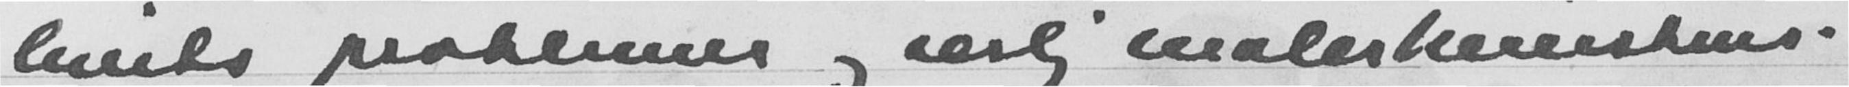livets problemer og særlig malerkunstens.
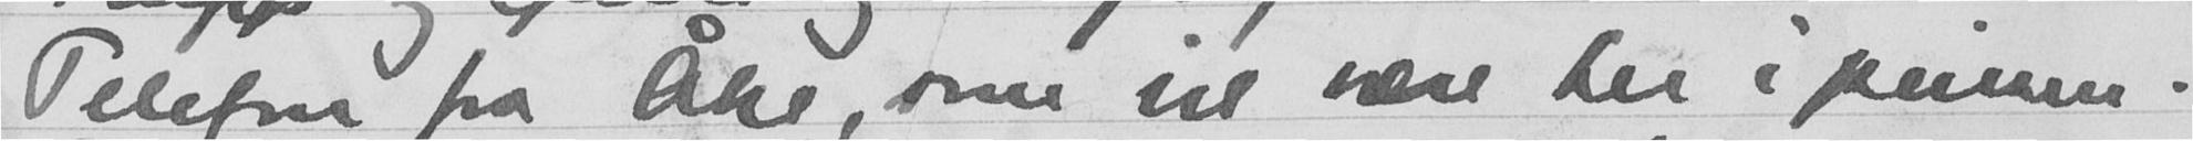Telefon fra Åke, som vil være her i pinsen.
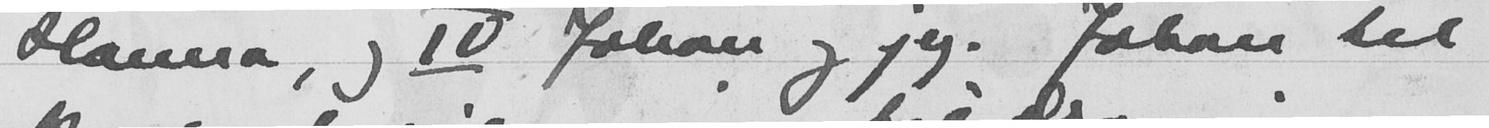Hanna, og IV Johan og jeg. Johan til
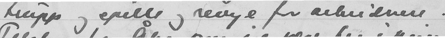trupp og spille og revye for arbeiderne.
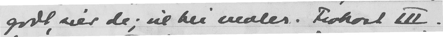godt, sier de: vil bli maler. Frokost III.
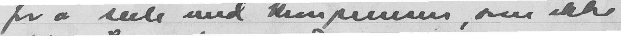for å seile med kronprinsen, som ikke
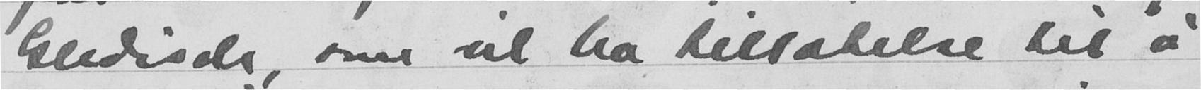Gledisch, som vil ha tillatelse til å
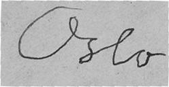Oslo
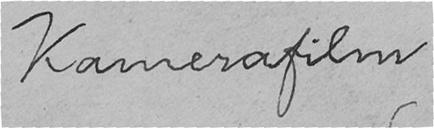Kamerafilm
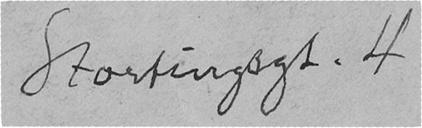Stortingsgt. 4
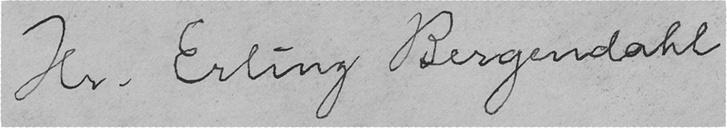Hr. Erling Bergendahl
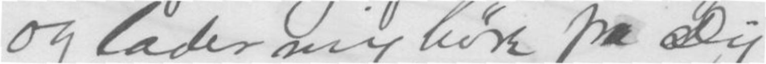og lader mig høre fra Dig
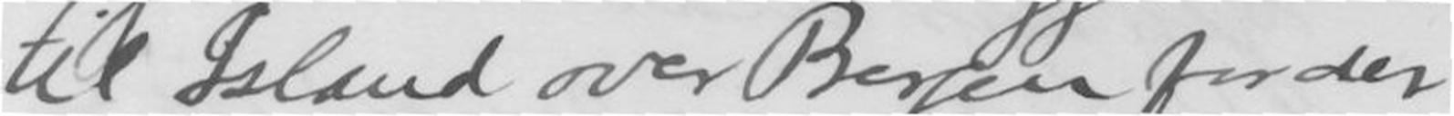til Island over Bergen for der
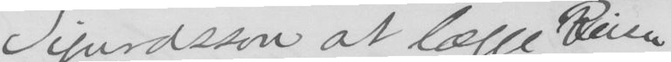Sigurdsson at lægge Reisen
